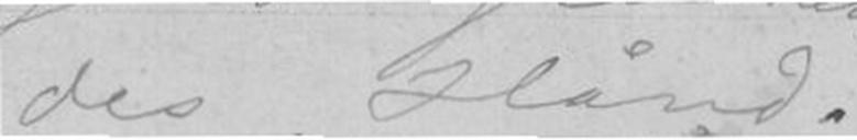des Hånd.
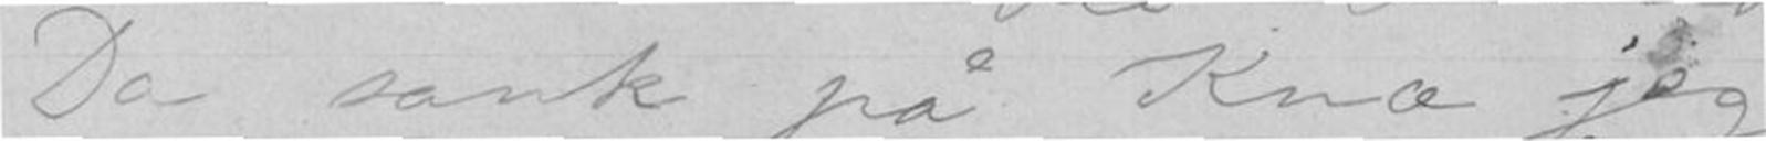Da sank på Knæ jeg
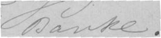Banke
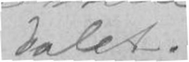talet.
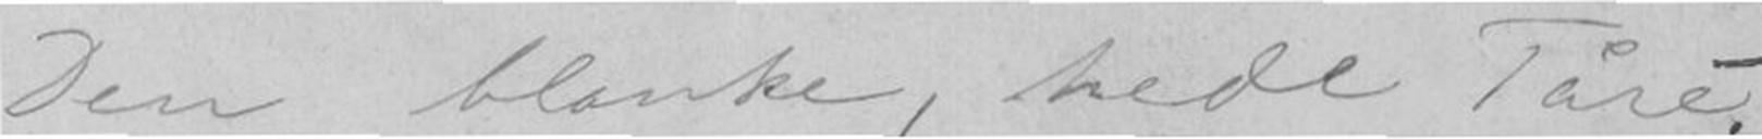Den blanke, hede Tåre. -
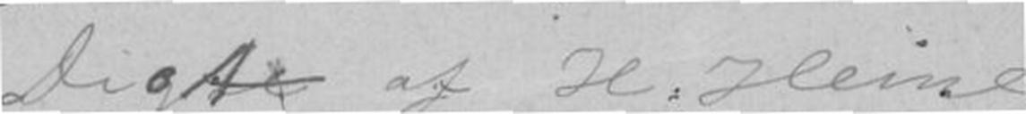Digte af H. Heime
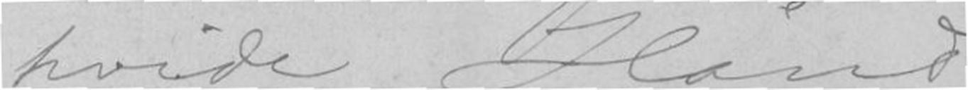hvide Hånd
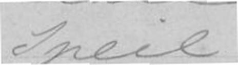Speil
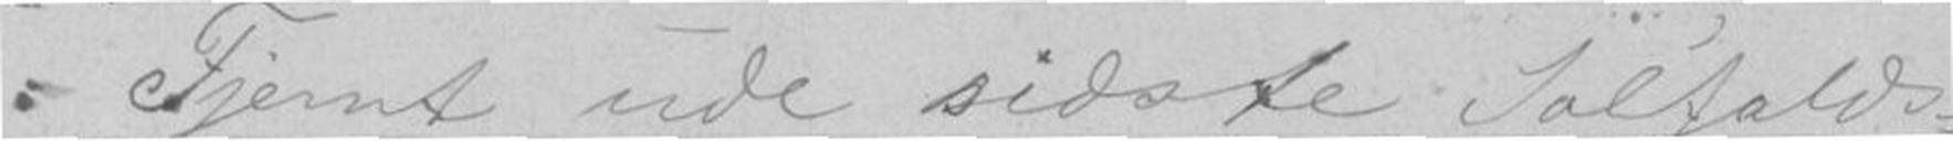Fjernt ude sidste Sol'falds-
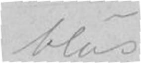blus
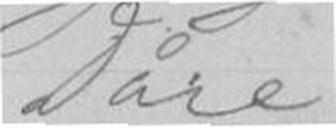Dåre,
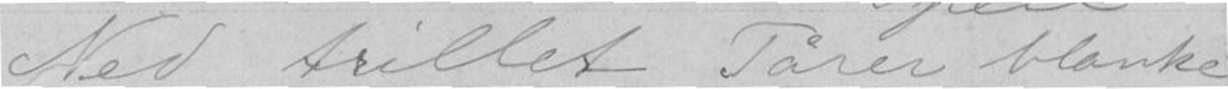Ned trillet Tårer blanke.
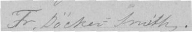Overs. ved af Fr. Döcker=Smith.
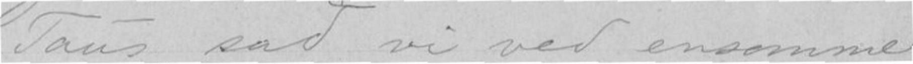Taus sad vi ved ensomme
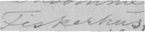Fiskerhus,
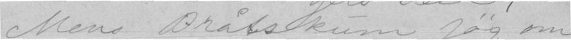Mens Bråtskum jøg om
